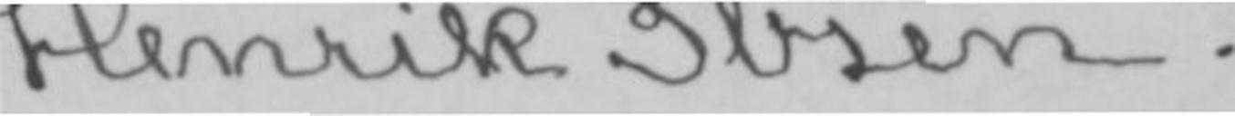Henrik Ibsen.
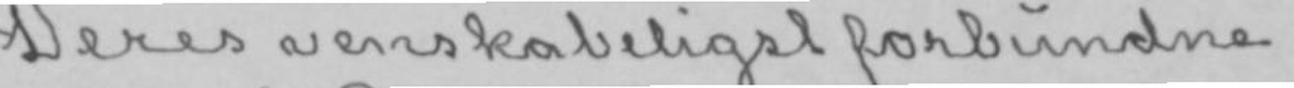Deres venskabeligst forbundne
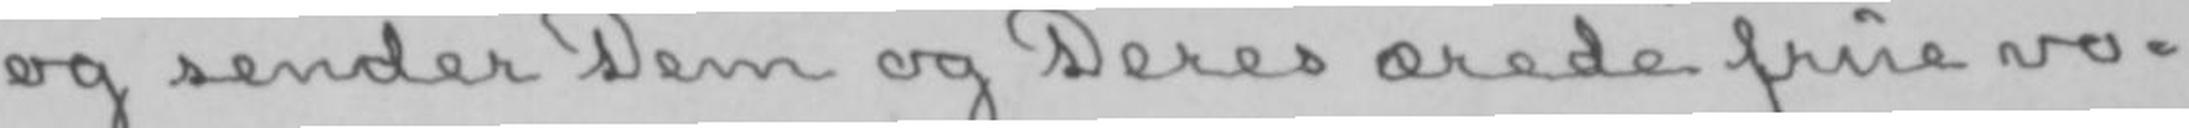og sender Dem og Deres ærede frue vo-
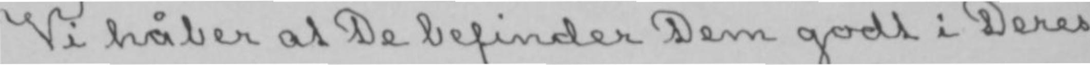Vi håber at De befinder Dem godt i Deres
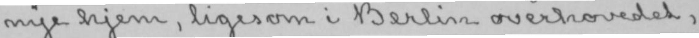nye hjem, ligesom i Berlin overhovedet,
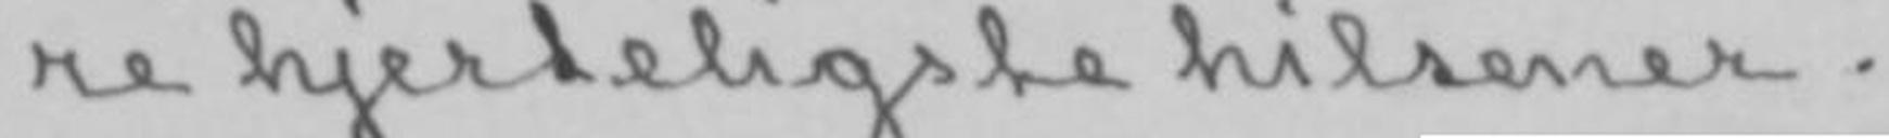re hjerteligste hilsener.
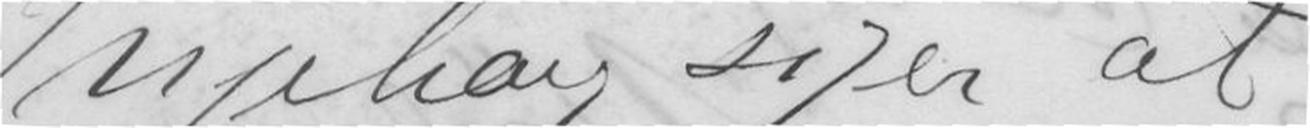Ingeborg siger at
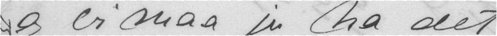og vi maa jo ha det
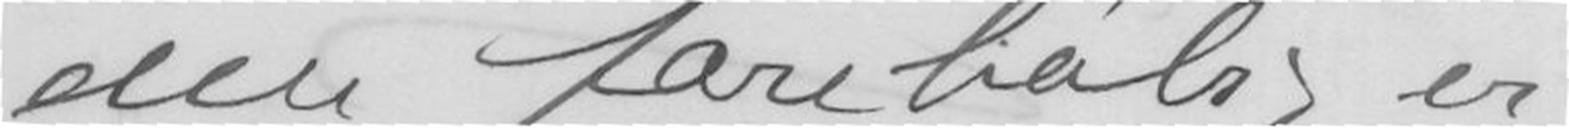den foreløbig er
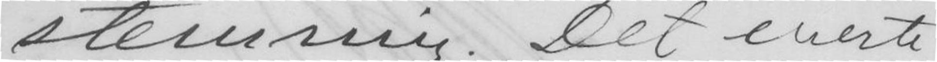stemning. Det eneste
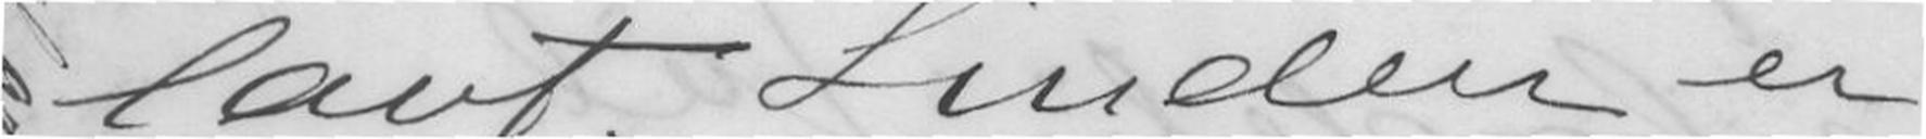lavt. Linden er
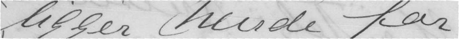ligger hende for
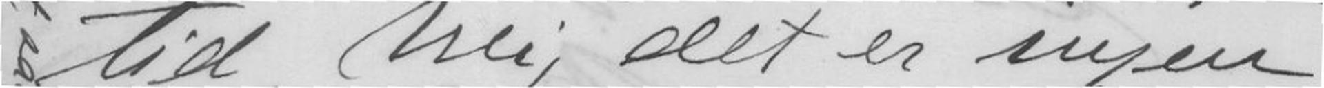tid. Nei, det er ingen
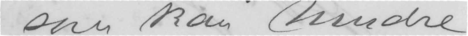som kan hindre
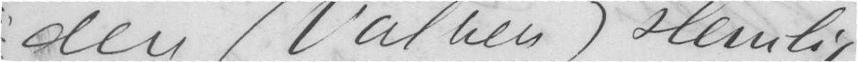den (Völven) stemlig
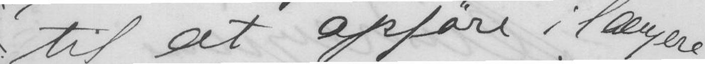til at opføre i længere
